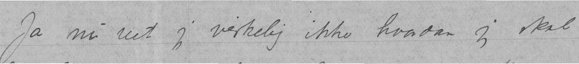Ja nu vet jeg virkelig ikke hvordan jeg skal
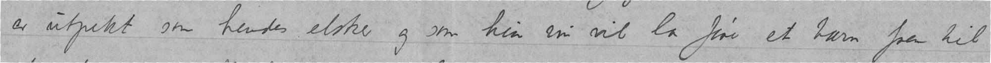er utpekt som hendes elsker og som hun nu vil la føre et barn frem til
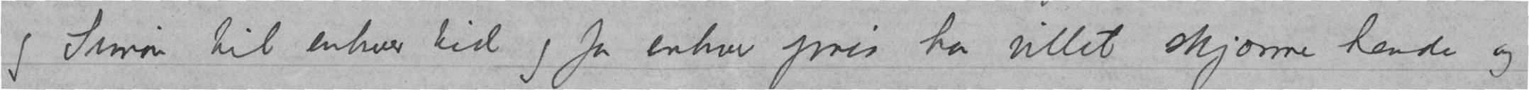og Simon til enhver tid og for enhver pris har villet skjærme hende og
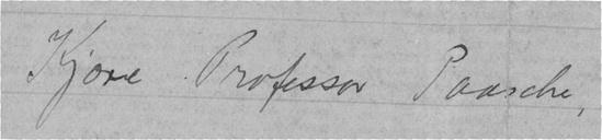Kjære Professor Paasche,
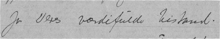for Deres værdifulde bistand.
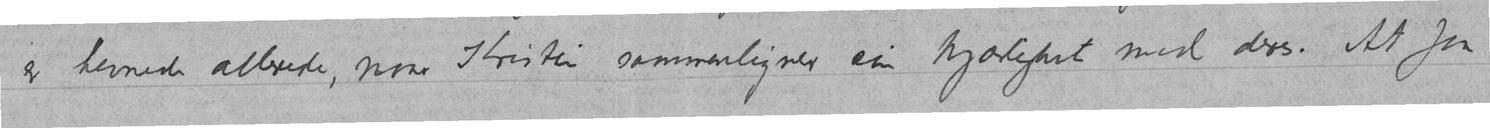er hevnede allerede, naar Kristin sammenligner sin kjærlighet med deres. At faa
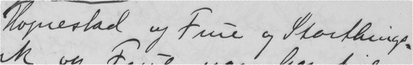Hognestad og Frue og Storthings-
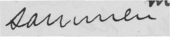sammen
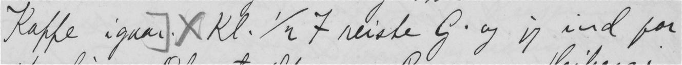Kaffe igaar.  Kl. 1/2 7 reiste G. og jeg ind for
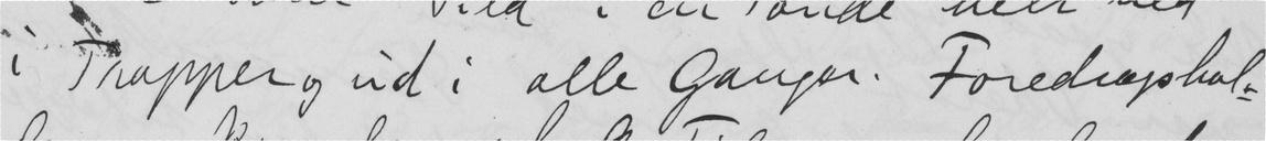i Trapper og ud i alle Gangar. Foredragshol-
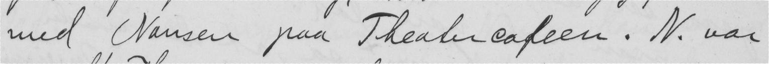med Nansen paa Theatercafeen. N. var
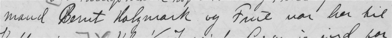mand Bernt Holzmark og Frue var her til
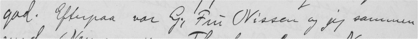god. Efterpaa var G., Fru Nissen og jeg sammen
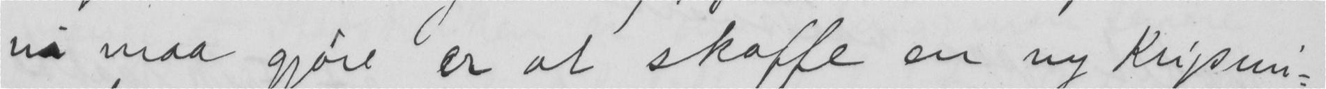vi maa gjøre er at skaffe en ny Krigsmi-
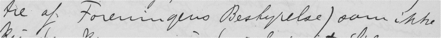tre af Foreningens Bestyrelse) som ikke
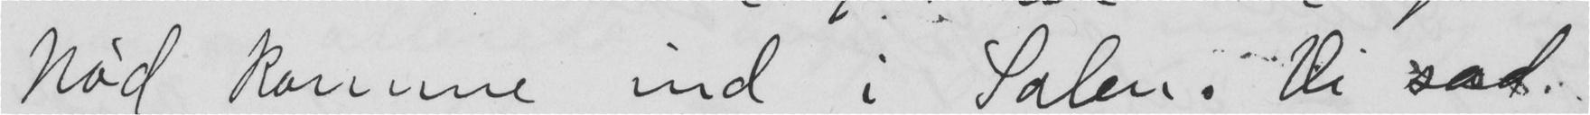Nød komme ind i Salen. Vi sad
